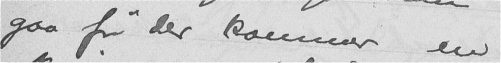gaa før der kommer en
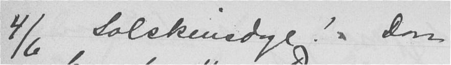4/6 Solskinsdage! - Don
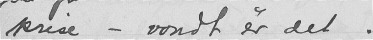krise - og vondt er det.
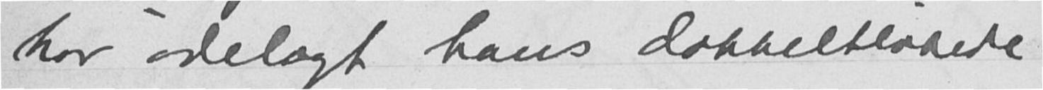har ødelagt hans dobbeltløbede
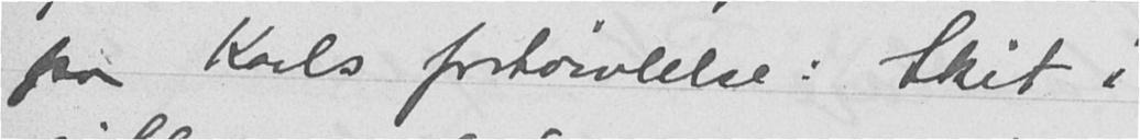paa Karls fortvivlelse: Skit i
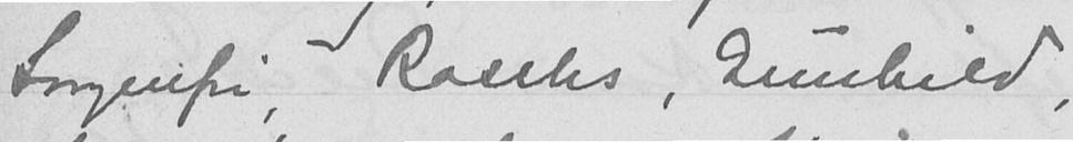Sorgenfri, Raschs, Gunhild,
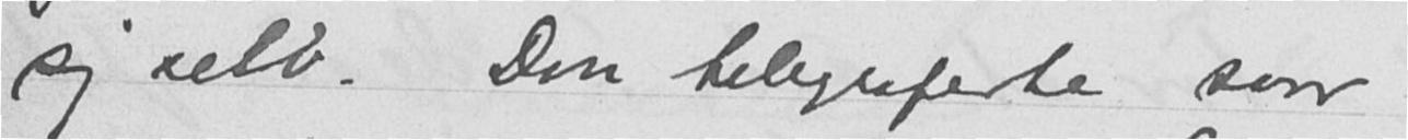sig selv. Don telegraferte svar
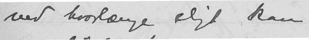ved hvorlænge sligt kan
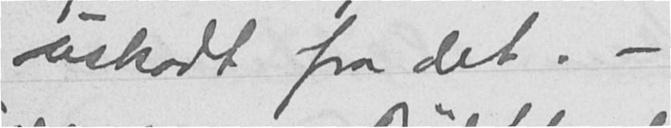uskadt fra det. -
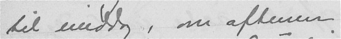til middag, om aftenen
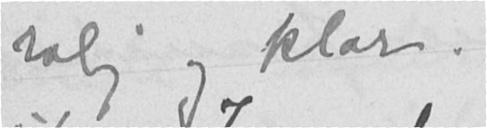rolig og klar.
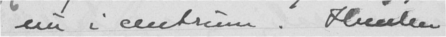nu i centrum. Himlen
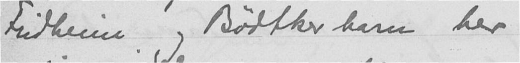Fridheim og Bødtker kom her
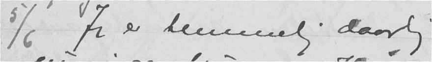5/6 Jeg er temmelig daarlig
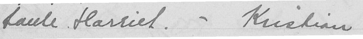tante Harriet. - Kristian
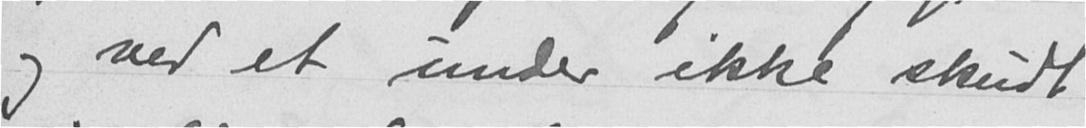og ved et under ikke skudt
